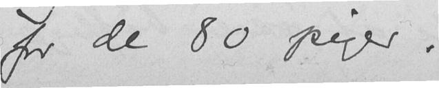for de 80 piger.
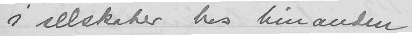i selskaber hos hinanden
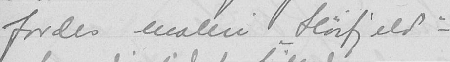Jordes maleri "Høifjeld" -
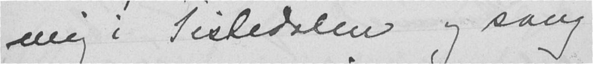mig i Tistedalen og sang
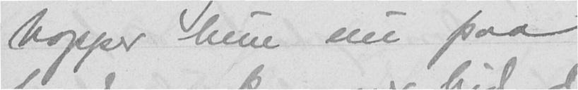hopper hun nu paa
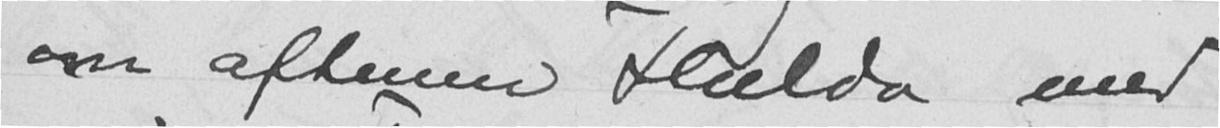om aftenen Hulda med
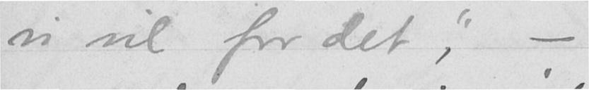vi vil for det," -
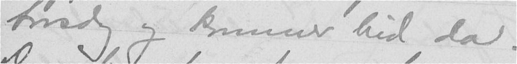torsdag og kommer hid da.
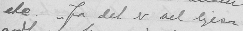etc. "Ja det er vel ligesaa
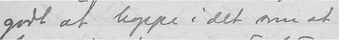godt at hoppe i det som at
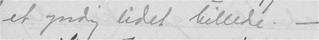et yndig lidet billede. -
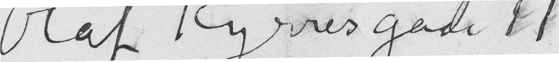Olaf Kyrres gade 11
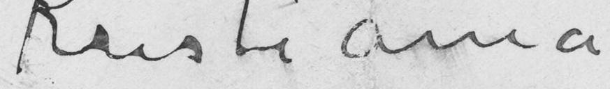Kristiania
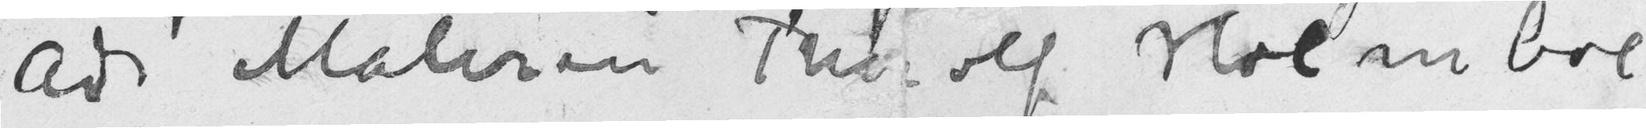Adr Maleren Thorolf Holmboe
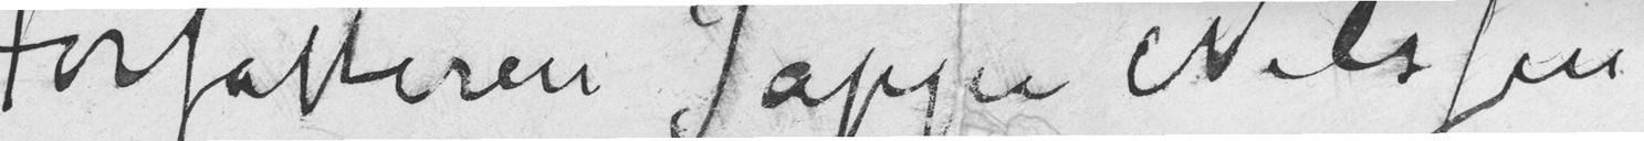Forfatteren Jappe Nilssen
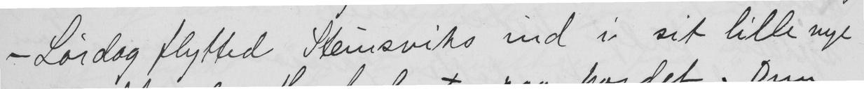- Lørdag flytted Steinsviks ind i sit lille nye
